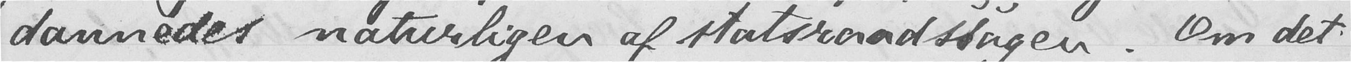dannedes naturligen af statsraadssagen. Om det
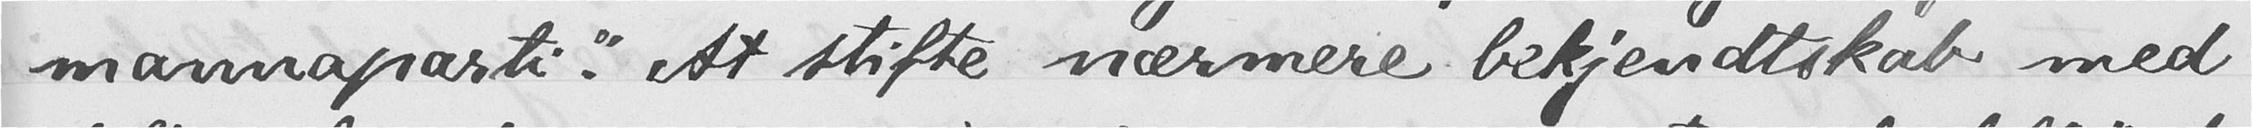mannaparti". At stifte nærmere bekjendtskab med
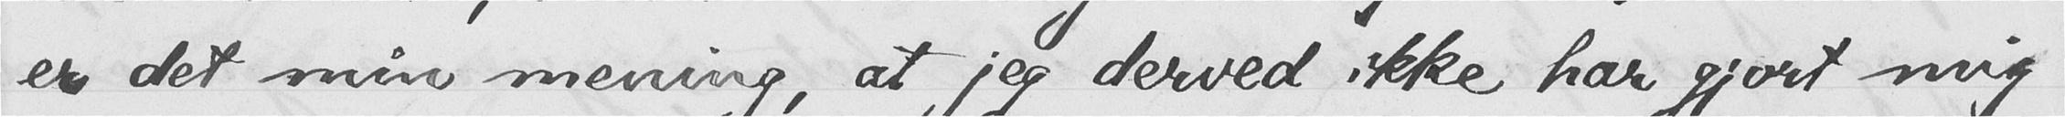er det min mening, at jeg derved ikke har gjort mig
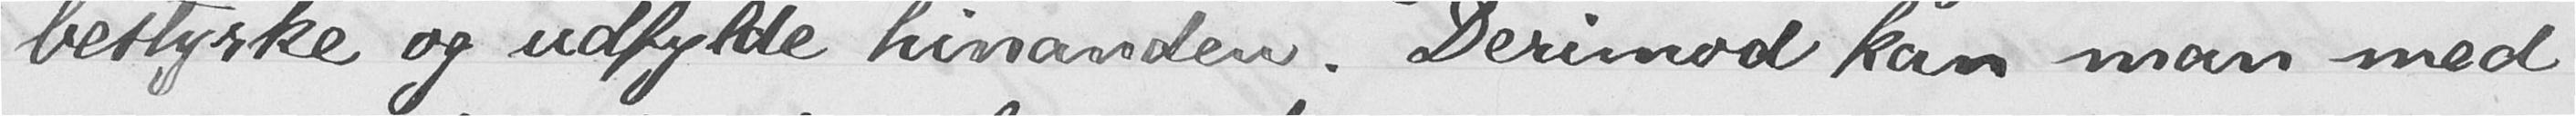bestyrke og udfylde hinanden. Derimod kan man med
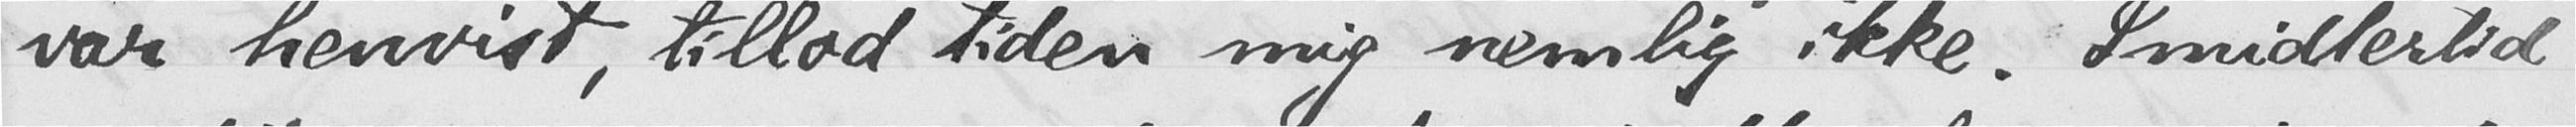var henvist, tillod tiden mig nemlig ikke. Imidlertid
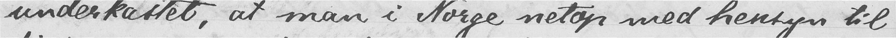underkastet, at man i Norge netop med hensyn til
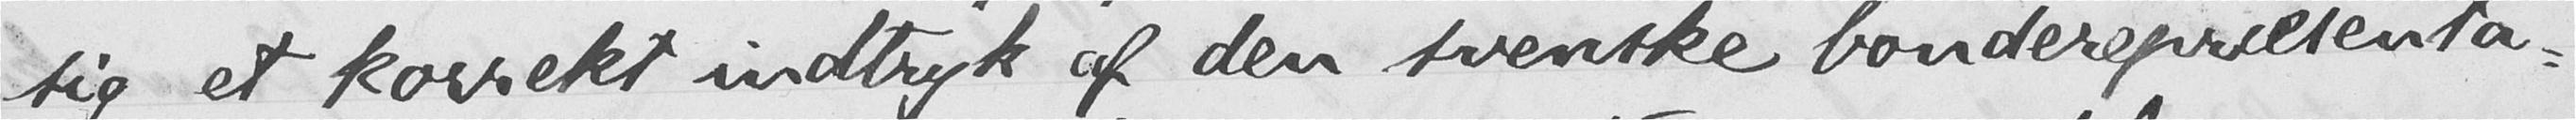sig et korrekt indtryk af den svenske bonderepræsenta-
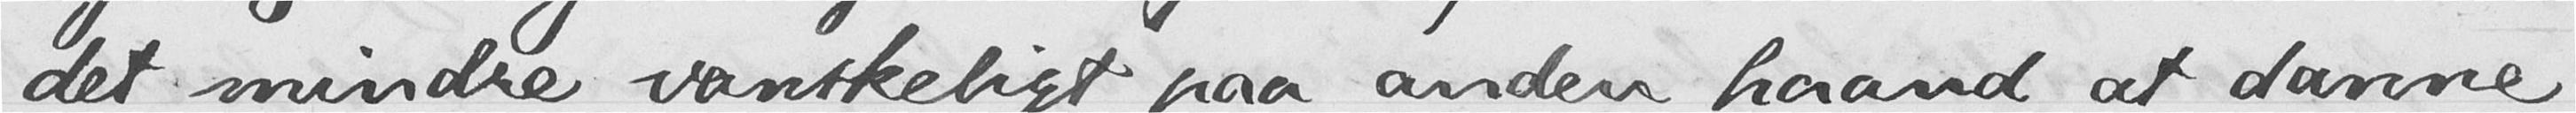det mindre vanskeligt paa anden haand at danne
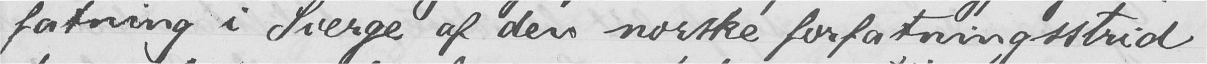fatning i Sverge af den norske forfatningsstrid
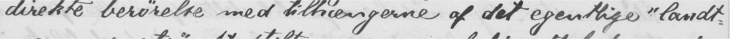direkte berørelse med tilhængerne af det egentlige "landt-
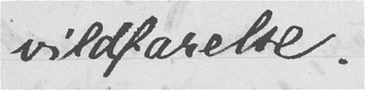vildfarelse.
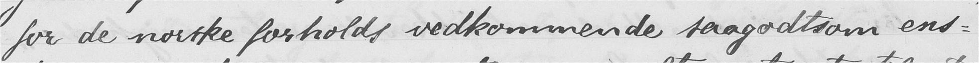for de norske forholds vedkommende saagodtsom ens-
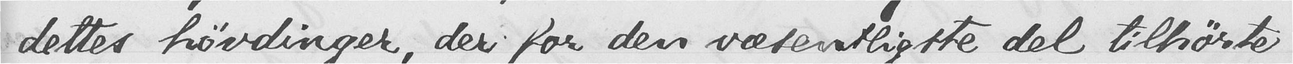dettes høvdinger, der for den væsentligste del tilhørte
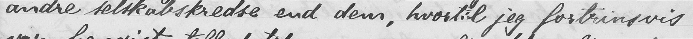andre selskabskredse end dem, hvortil jeg fortrinsvis
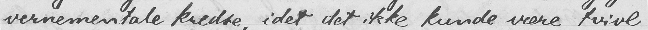vernementale kredse, idet det ikke kunde være tvivl
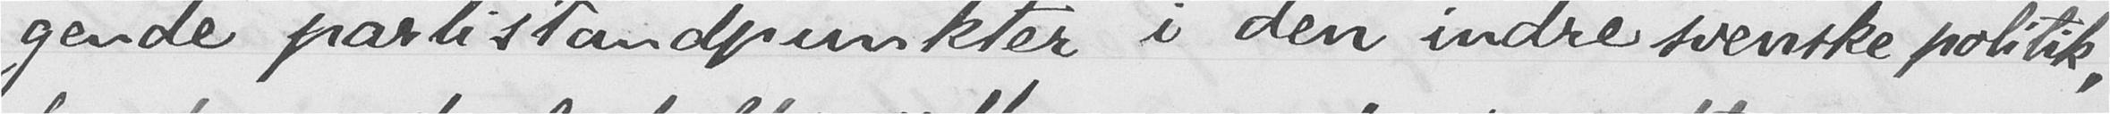gende partistandpunkter i den indre svenske politik,
